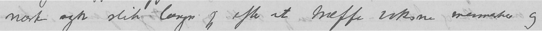næste syk slik længes jeg efter at træffe voksne mennesker og
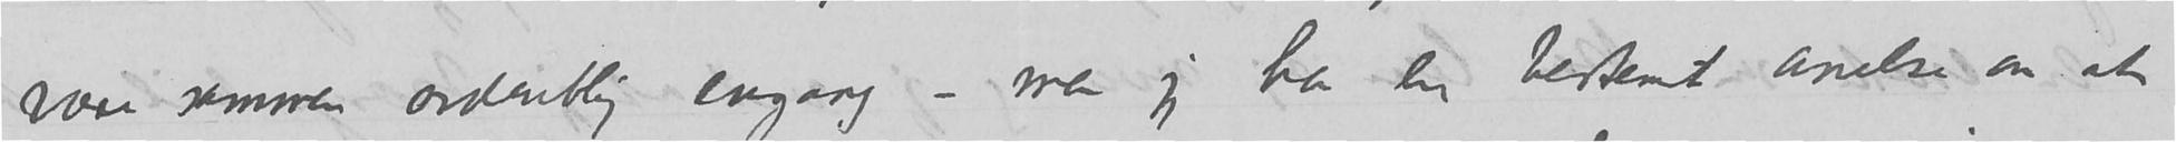være sammen ordentlig engang – men jeg har en bestemt anelse om at
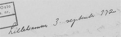Lillehammer 3. september 1920
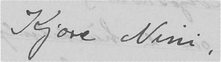Kjære Nini,
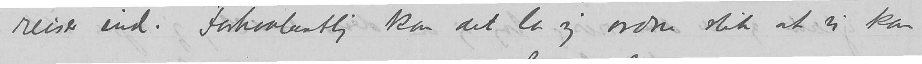reiser ind. Forhaabentlig kan det la sig ordne slik at vi kan
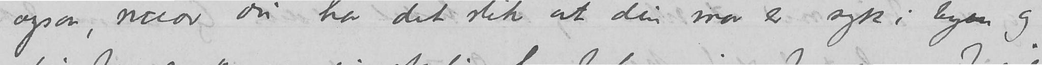ogsaa, naar du har det slik at din mor er syk i byen og
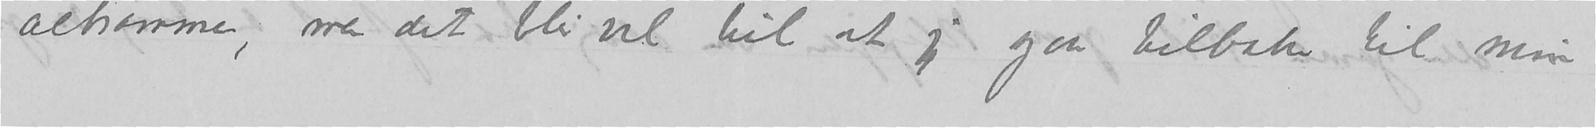altsammen, men det blir vel til at jeg gaar tilbake til min
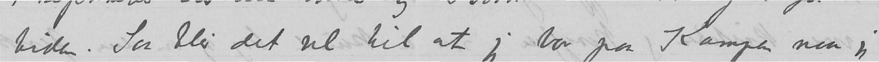tiden. Saa blir det vel til at jeg bor paa Kampen naar jeg
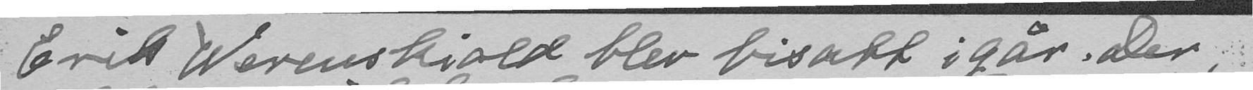Erik Werenskjold blev bisatt igår. Der
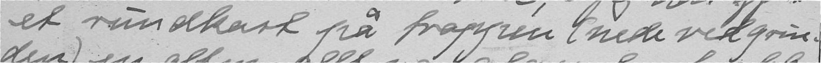et rundkast på trappen (nede ved grin-
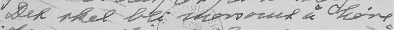Det skal bli morsomt å høre
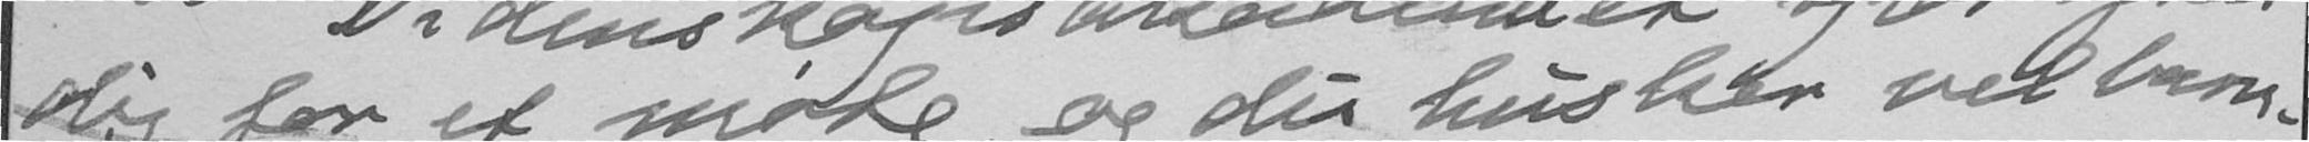dig for et møte, og du husker vel barn-
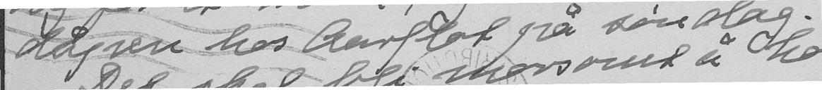dåpen hos Aarflot på søndag!
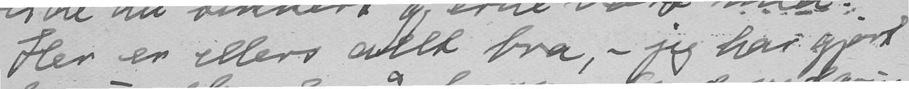Her er ellers allt bra, - jeg har gjort
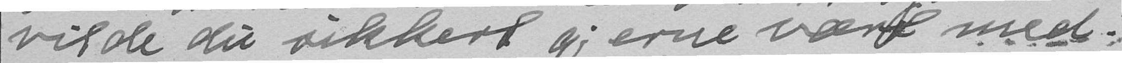vilde du sikkert gjerne vært med!
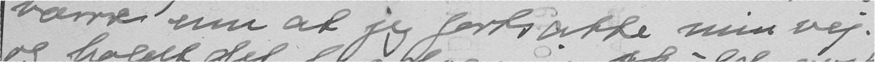værre enn at jeg fortsatte min vej
